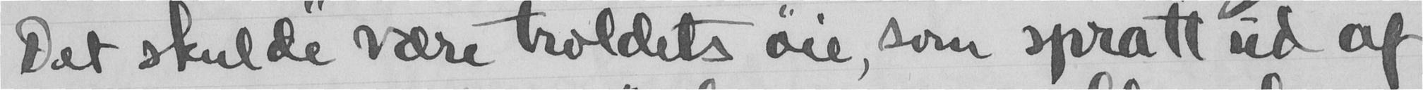Det skulde være troldets øie, som spratt ud af
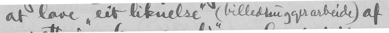at lave eit liknelse (billedhuggerarbeide) af
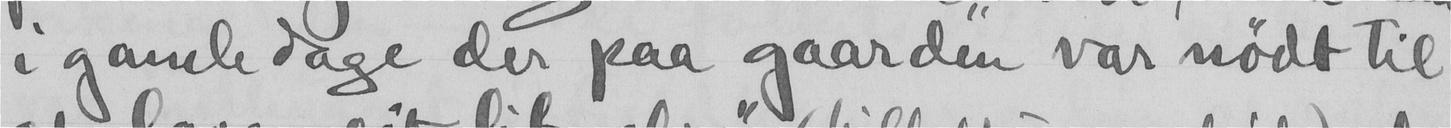i gamle dage der paa "gaarden" var nødt til
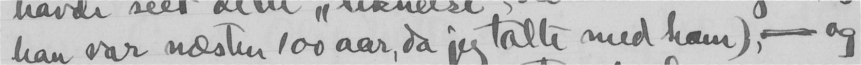han var næsten 100 aar, da jeg talte med ham), - og
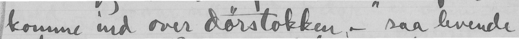komme ind over dørstokken, - saa levende
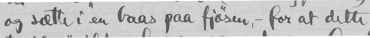og sætte i en baas paa fjøsen, - for at dette
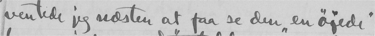ventede jeg næsten at faa se den "en øjede"
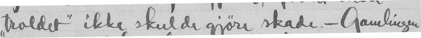"troldet" ikke skulde gjøre skade. - Gamlingen
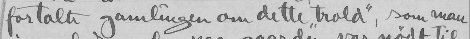fortalte gamlingen om dette "trold", som man
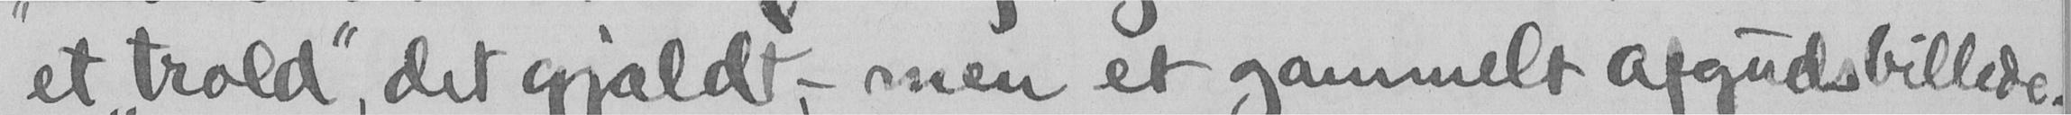et "trold", det gjaldt, - men et gammelt afgudsbillede.
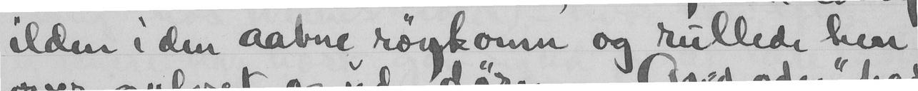ilden i den aabne røykomn og rullede hen
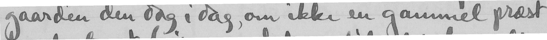gaarden den dag i dag, om ikke en gammel præst
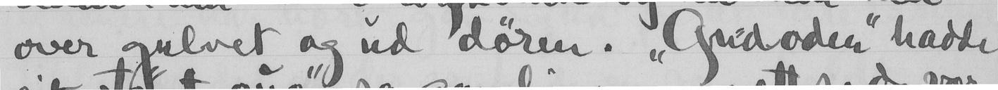over gulvet og ud døren! "Gu-doden" hadde
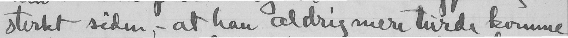sterkt siden, - at han aldrig mere turde komme
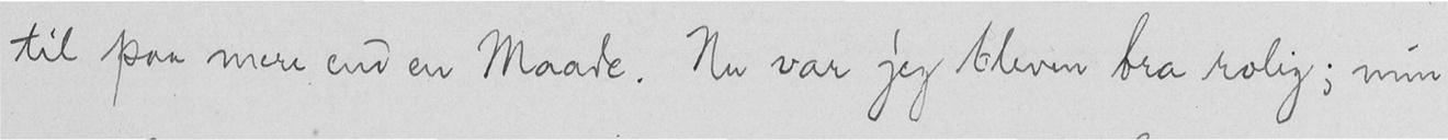til paa mere end en Maade. Nu var jeg bleven bra rolig; min
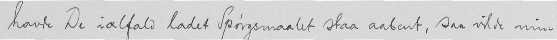havde De ialfald ladet Spørgsmaalet staa aabent, saa vilde min
